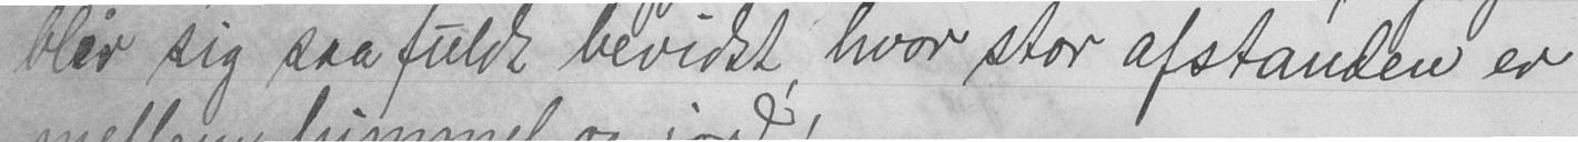blev sig saa fuldt bevidst hvor stor afstanden er
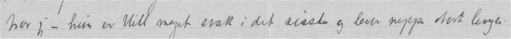tror jeg – hun er blitt meget svak i det sisste og lever neppe stort lenger.
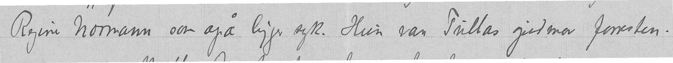Regine Normann som ogå ligger syk. Hun var Tullas gudmor forresten.
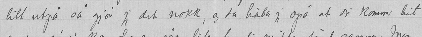litt utpå så gjør jeg det nokk, og da håber jeg også at du kommer hit
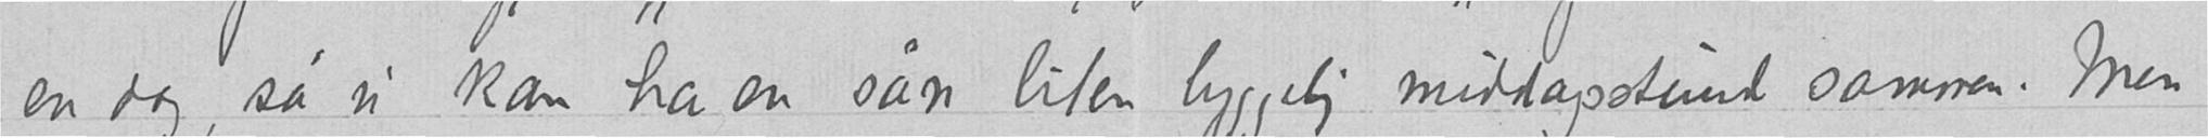en dag, så vi kan ha en sån liten hyggelig middagsstund sammen. Men
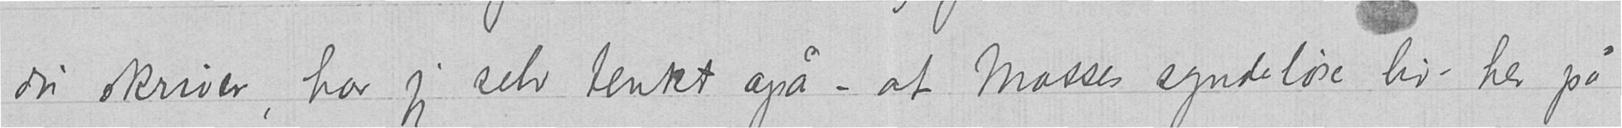du skriver, har jeg selv tenkt også – at Mosses syndeløse liv her på
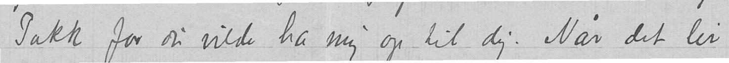Takk for du vilde ha mig op til dig. Når det lir
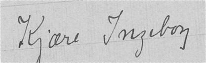Kjære Ingeborg
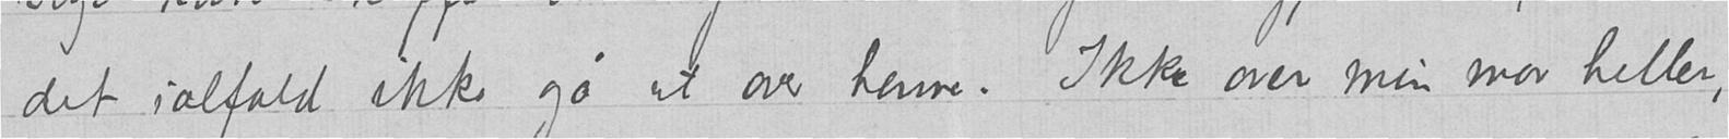det ialfald ikke gå ut over henne. Ikke over min mor heller,
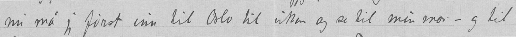nu må jeg først inn til Oslo til uken og se til min mor - og til
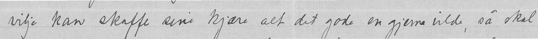vilje kan skaffe sine kjære alt det gode en gjerne vilde, så skal
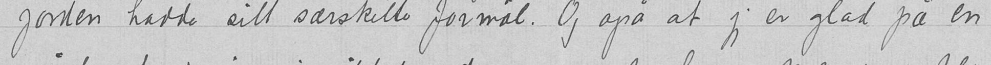jorden hadde sitt særskilte formål. Og også at jeg er glad på en
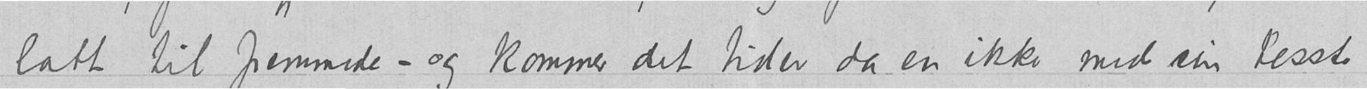latt til fremmede - og kommer det tider da en ikke med sin besste
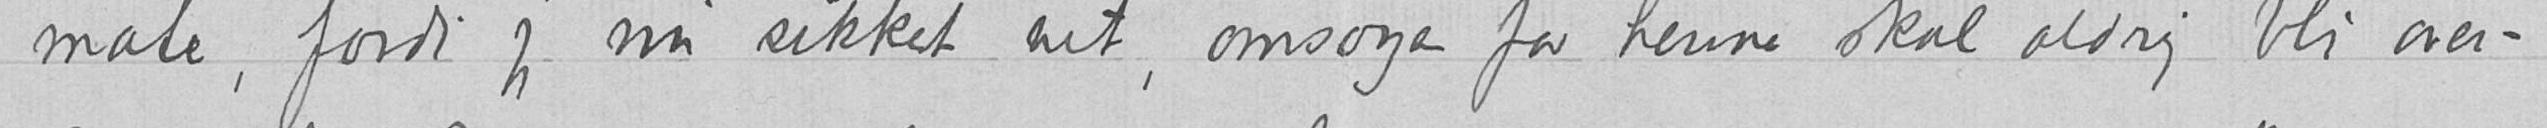måte, fordi jeg nu sikkert vet, omsorgen for henne skal aldrig bli over-
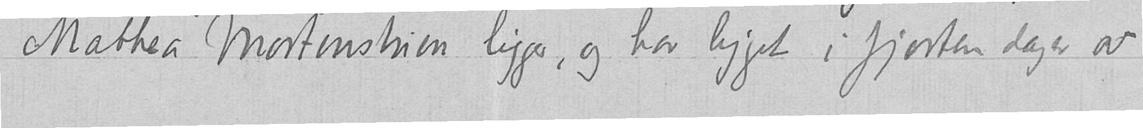Mathea Mortenstuen ligger, og har ligget i fjorten dager av
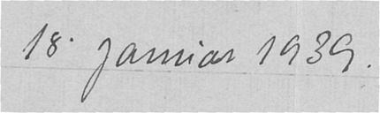18. januar 1939.
